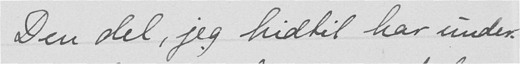Den del, jeg hidtil har under-
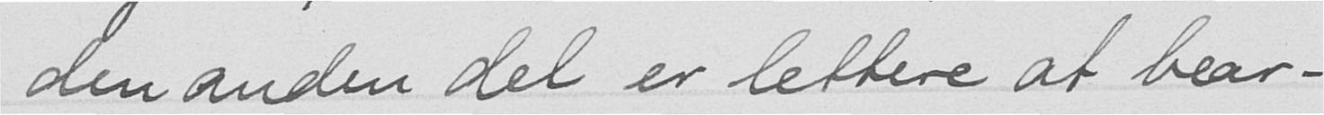den anden del er lettere at bear-
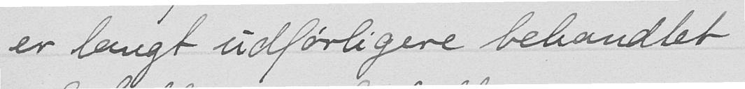er langt udførligere behandlet
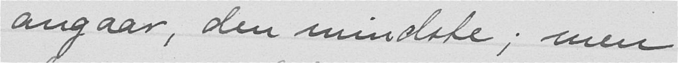angaar, den mindste, men
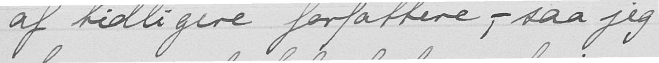af tidligere forfattere, - saa jeg
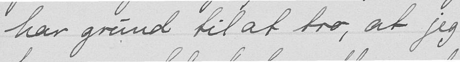har grund til at tro, at jeg
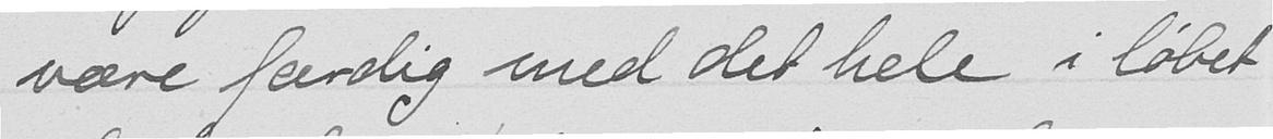være færdig med det hele i løbet
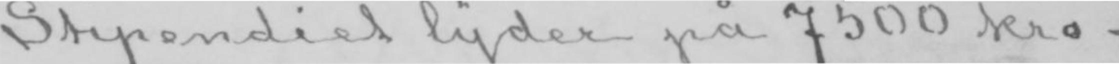Stipendiet lyder på 7500 kro-
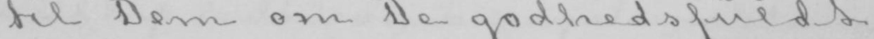til Dem om De godhedsfuldt
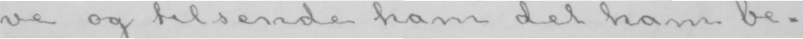ve og tilsende ham det ham be-
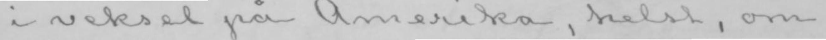i veksel på Amerika, helst, om
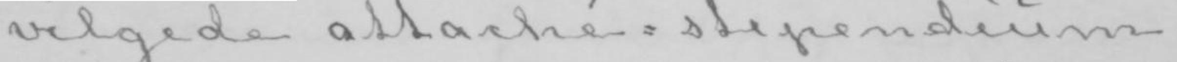vilgede attaché-stipendium.
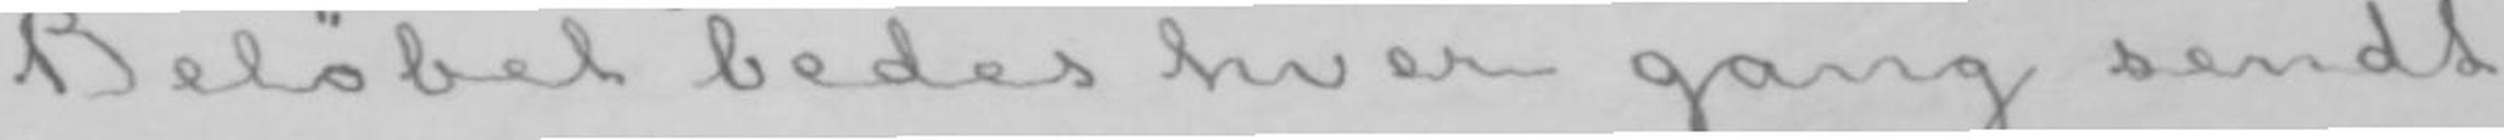Beløbet bedes hver gang sendt
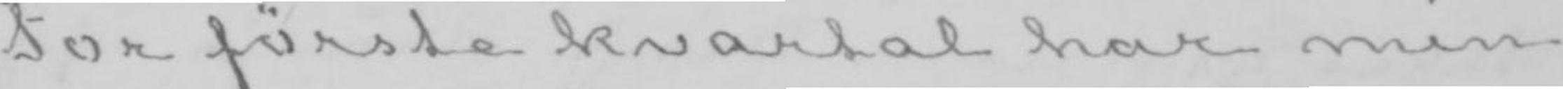For første kvartal har min
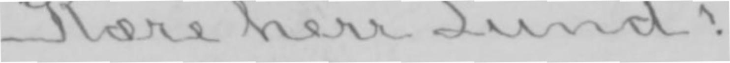Kære herr Lund!
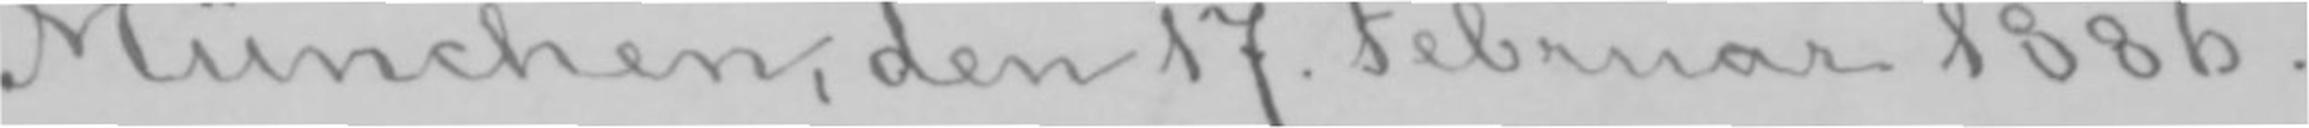München, den 17. Februar 1886.
